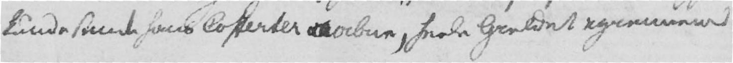kunde sende hans Cofferter aabne, heele Gieldet igiennem
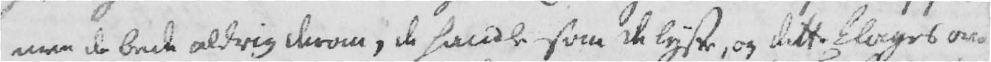erne de bede aldrig derom, de handle som de lyste, og dette Klages over
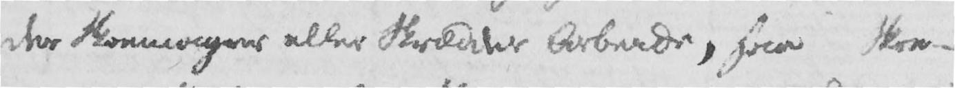der Skoemagers eller Skrædders Arbeide, han Skoe-
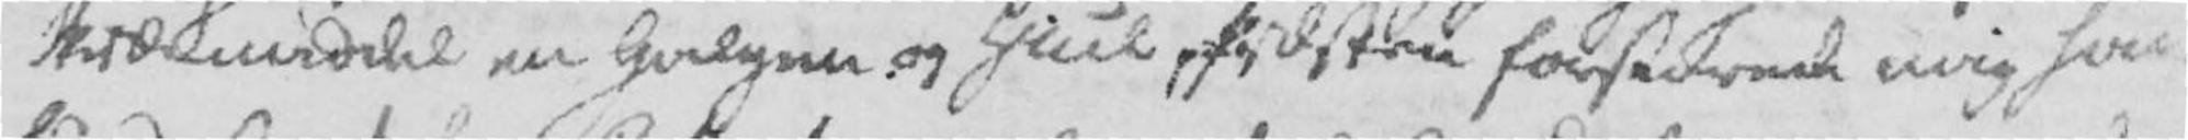Skrækmiddel en Galgen og Hiul, Præsten forsikrede mig han
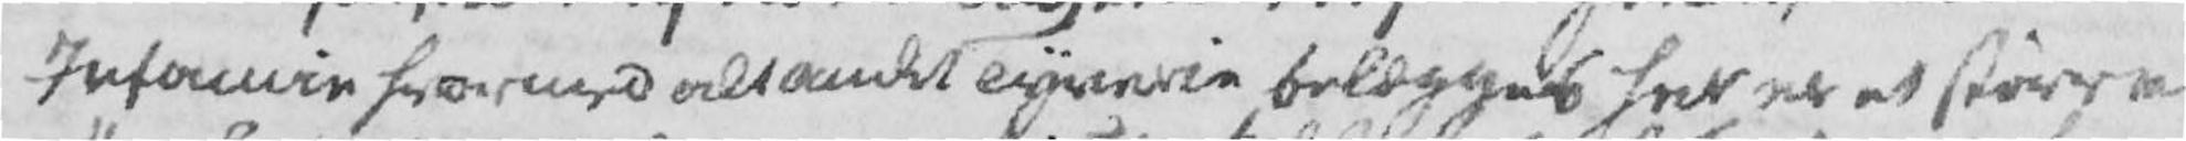Infamie hvormed alt andet Tyverie belægges her er et større
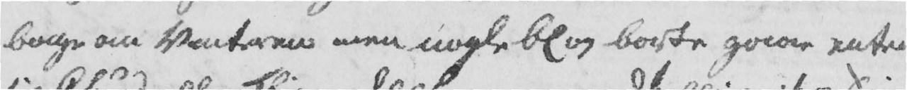bage om Vinteren men nogle bl og borte gaae enten
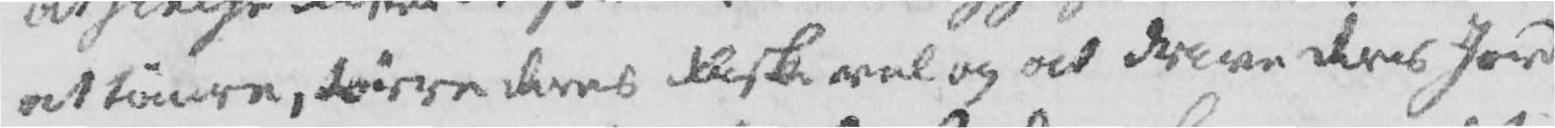at tømre, tørre deres Fiskevel og at drive deres Jord-
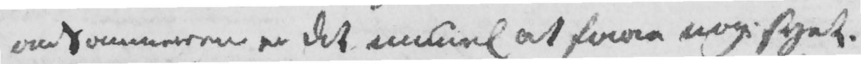om Sommeren er det umuel at faae nog. syet.
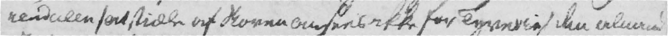rendalen / at stiæle af Skoven ansees ikke for Tyverie / den almind
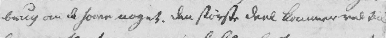brug om de have noget. Den største Deel kommer vel til-
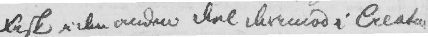Fisk i den anden del derimod i Creatur.
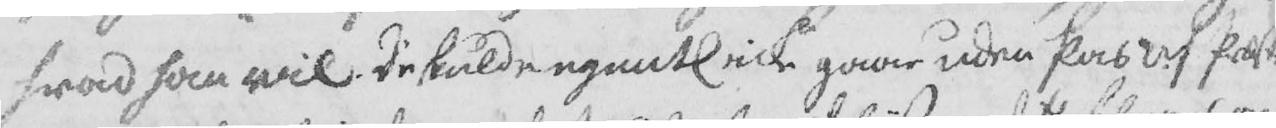hvad han vil. De skulde egentl icke gaae uden Pas af Præst-
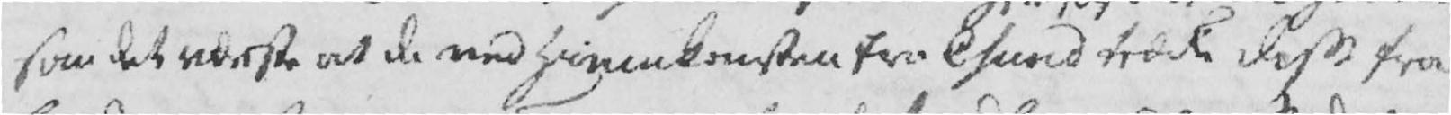som det værste at de ved Hiemkomsten fra Csund træcke disse fra
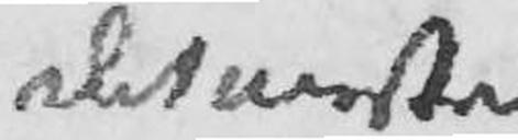det meste
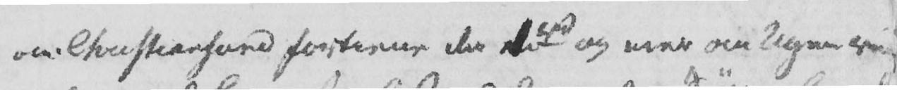om Christiansund fortiene de 1 rd og mer om Ugen ved
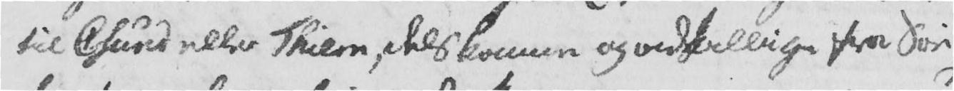til Csund eller Thiem, dels komme og adskillige fra Søe-
